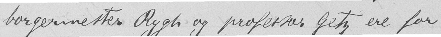borgermester Rygh og professor Getz ere for
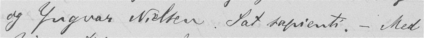og Yngvar Nielsen. Sat sapienti. – Med
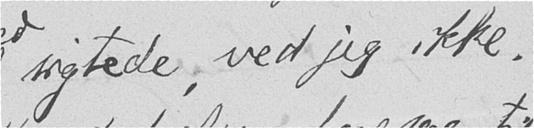sigtede, ved jeg ikke.
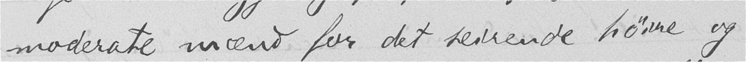moderate mænd for det seirende høire og
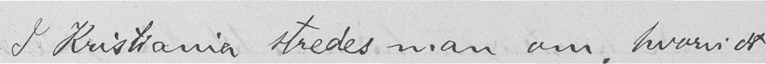I Kristiania stredes man om, hvorvidt
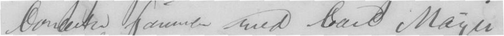Concerter sammen med Carl Mayer,
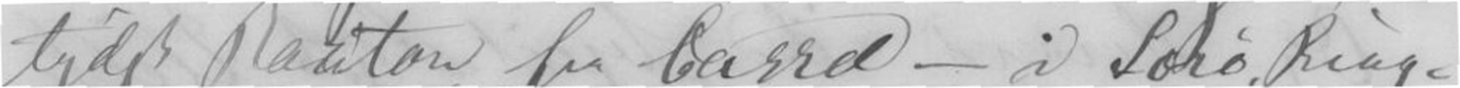tydsk Bariton fra Cassel - i Lærø, Rung-
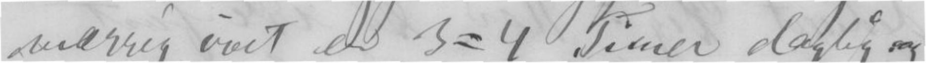mæssig øvet en 3=4 Timer daglig og
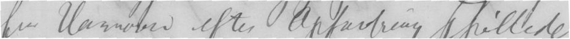fra Hannover efter Opfordring spillede
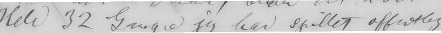Hele 32 Gange jeg har spillet offentlig,
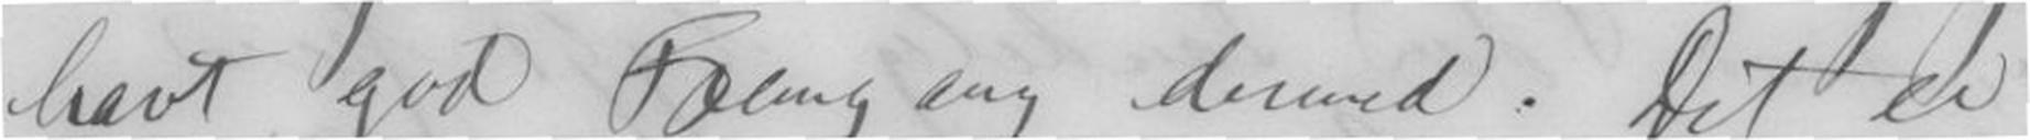havt god Fræmgang dermed. Det er
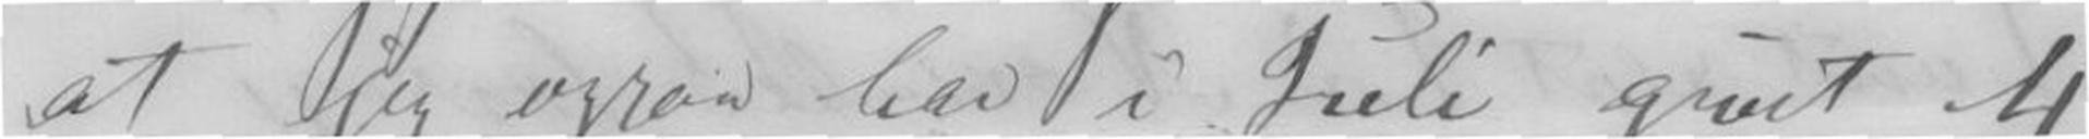at jeg ogsaa har i Juli givet 4
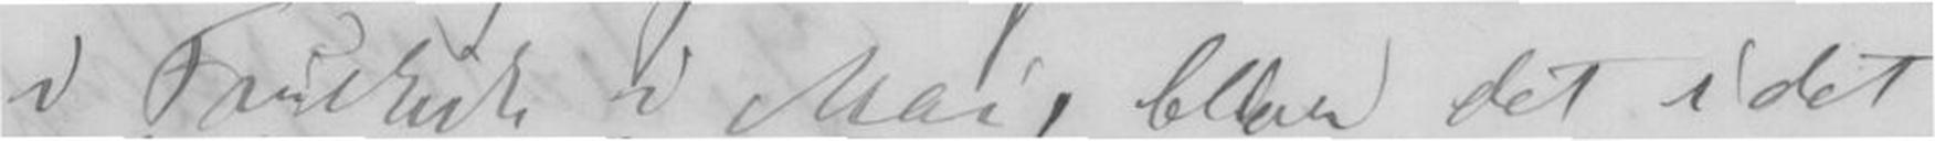i Frankrike i Mai, bliver det i det
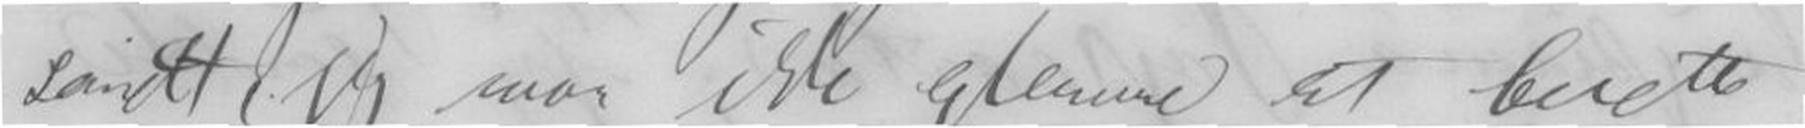sandt jeg maa ikke glemme at berette,
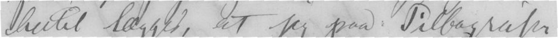hertil lægges, at jeg paa Tilbagereisen
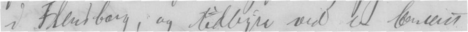i Flensburg, og tidligere ved en Concert
